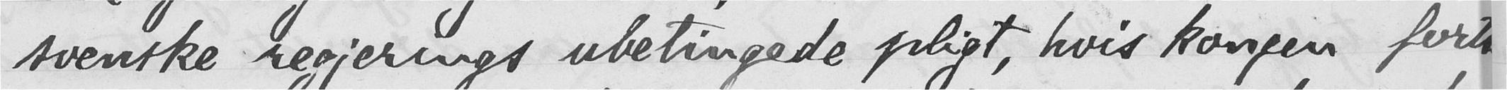svenske regjerings ubetingede pligt, hvis kongen fortsatte
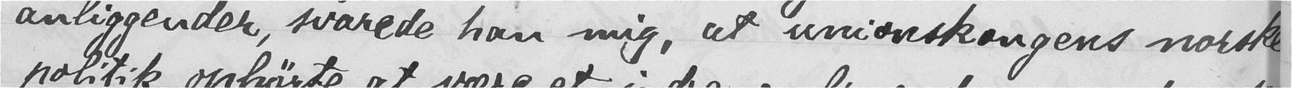anliggender, svarede han mig, at unionskongens norske
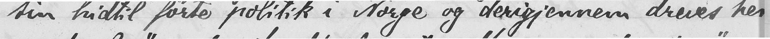sin hidtil førte politik i Norge og derigjennem dreves heni-
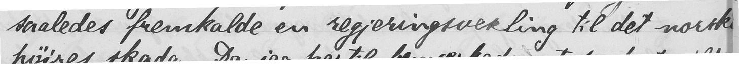saaledes fremkalde en regjeringsvexling til det norske
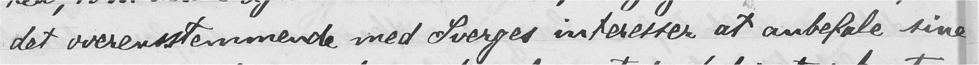det overensstemmende med Sverges interesser at anbefale sine
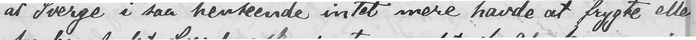at Sverge i saa henseende intet mere havde at frygte eller
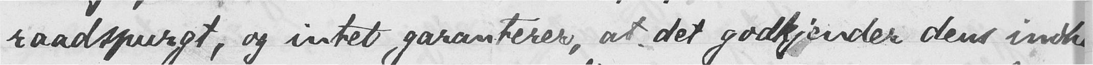raadspurgt, og intet garanterer, at det godkjender dens indhold."
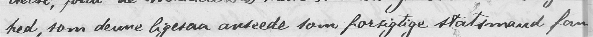hed, som denne ligesaa anseede som forsigtige statsmand fandt
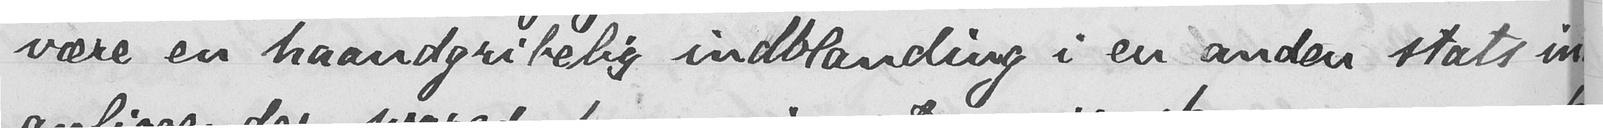være en haandgribelig indblanding i en anden stats indre
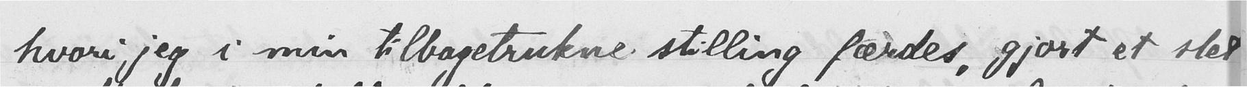hvori jeg i min tilbagetrukne stilling færdes, gjort et slet
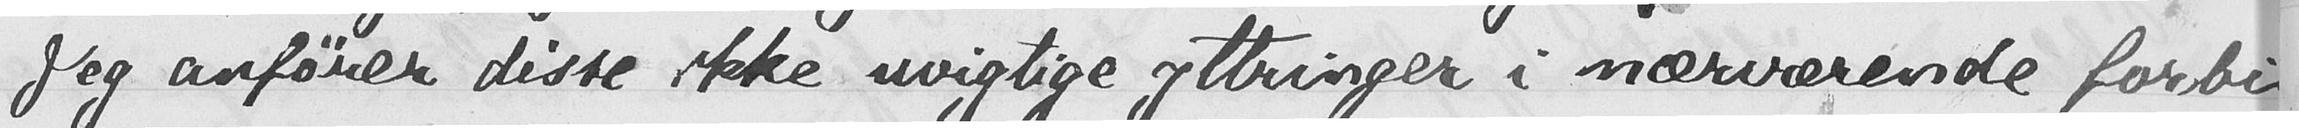Jeg anfører disse ikke uvigtige yttringer i nærværende forbin-
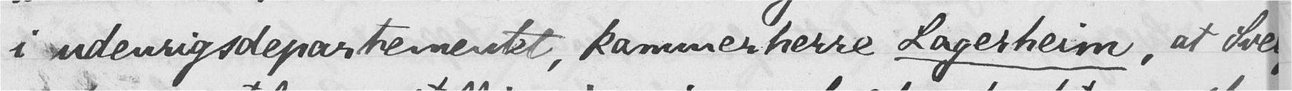i udenrigsdepartementet, kammerherre Lagerheim, at Sverge
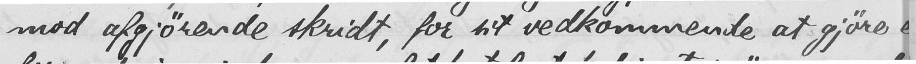mod afgjørende skridt, for sit vedkommende at gjøre en
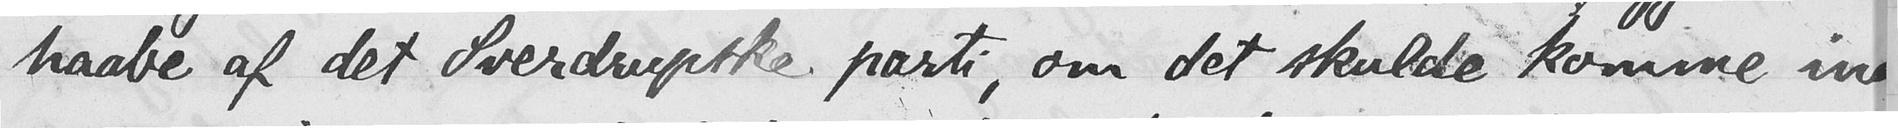haabe af det Sverdrupske parti, om det skulde komme ind
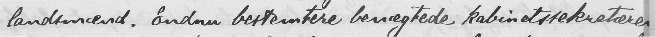landsmænd. Endnu bestemtere benægtede kabinetssekretæren
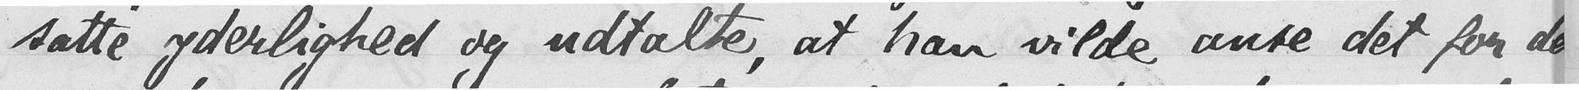satte yderlighed og udtalte, at han vilde anse det for den
